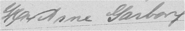Hr Arne Garborg
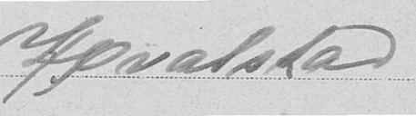Hvalstad.
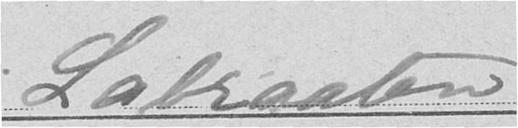Labraaten
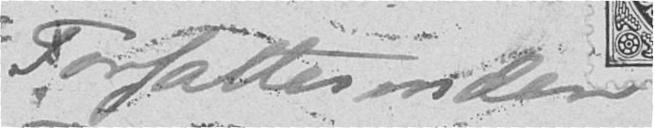Forfatterinden
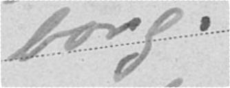borg.
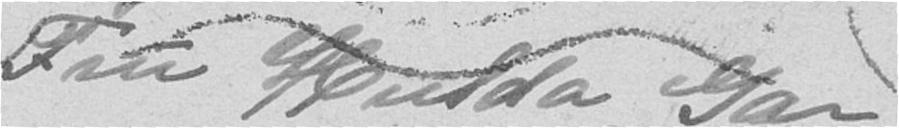Fru Hulda Gar-
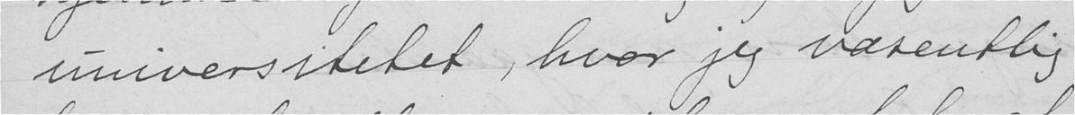universitetet, hvor jeg væsentlig
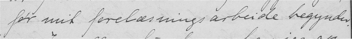før mit forelæsningsarbeide begynder.
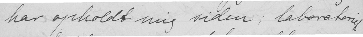har opholdt mig siden; laboratoriet
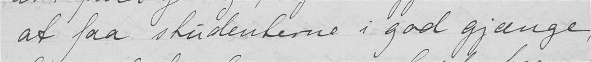at faa studenterne i god gjænge,
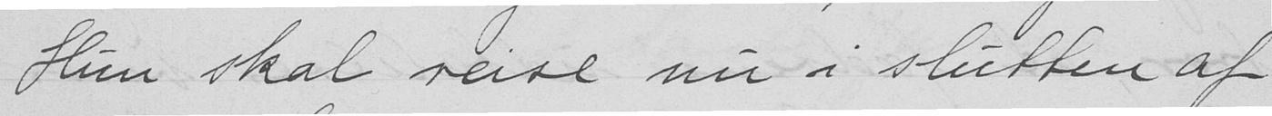Hun skal reise nu i slutten af
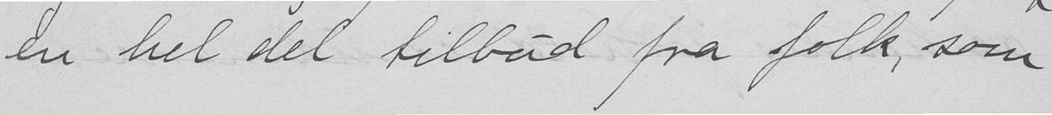en hel del tilbud fra folk, som
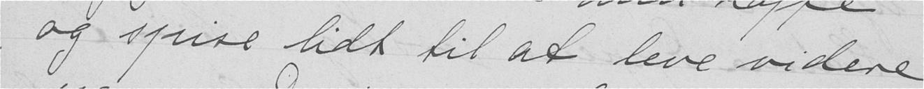og spise lidt til at leve videre
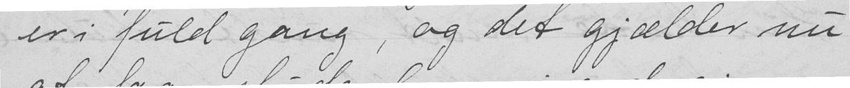er i fuld gang, og det gjælder nu
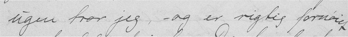ugen tror jeg, - og er rigtig fornøiet
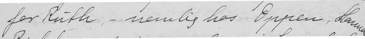for Ruth, - nemlig hos Oppen, Hanna
